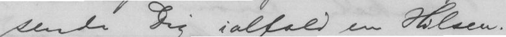sende Dig ialfald en Hilsen.
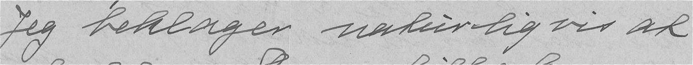Jeg beklager naturligvis at
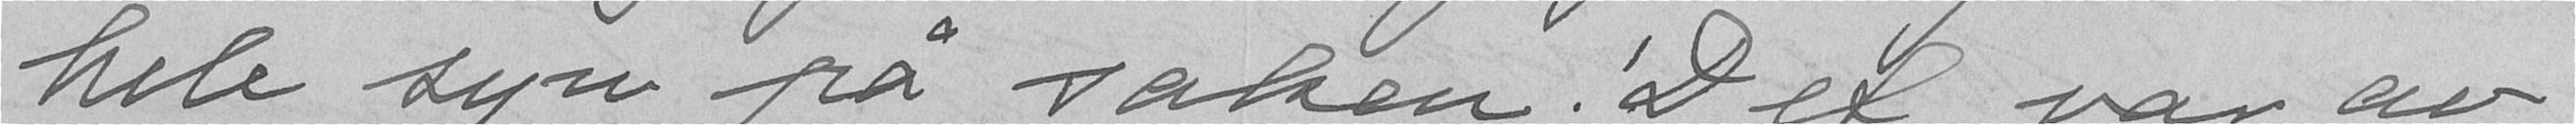hele syn på saken. Det var av
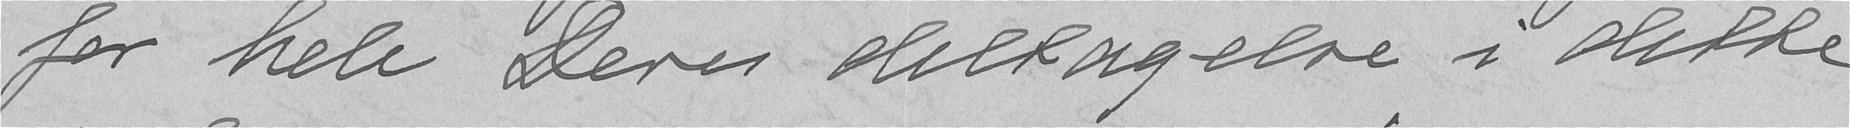for hele Deres deltagelse i dette
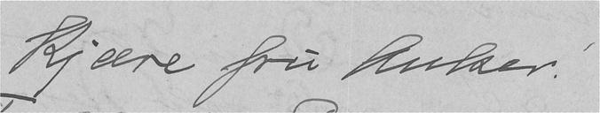Kjære fru Anker!
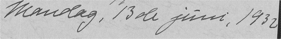Mandag, 13de juni, 1932
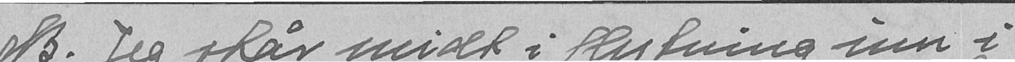NB. Jeg står midt i flytning inn i
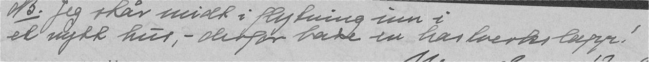et nytt hus, - derfor bare en hastverkslapp!
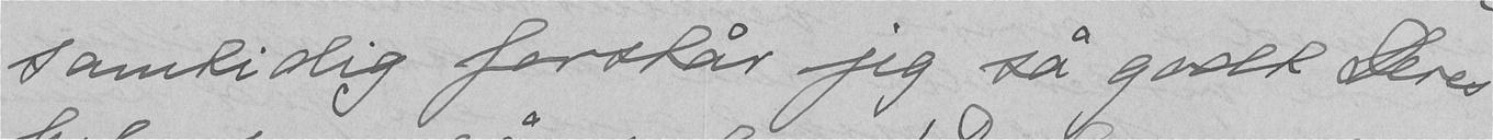samtidig forstår jeg så godt Deres
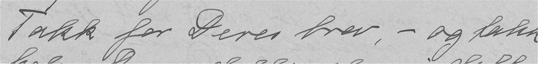Takk for Deres brev, - og takk
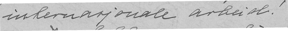internasjonale arbeid!
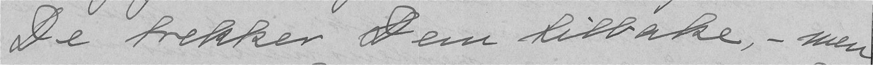De trekker Dem tilbake, - men
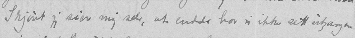Skjønt jeg sier mig selv, at endda har vi ikke sett utgangen
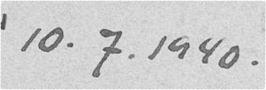10.7.1940.
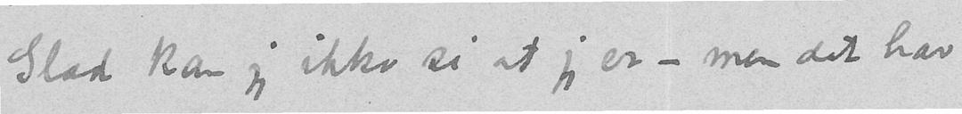Glad kan jeg ikke si at jeg er - men det har
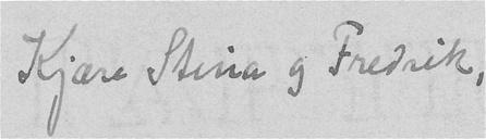Kjære Stina og Fredrik,
